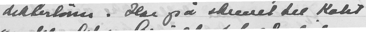dikterlønn. Har også skrevet til Koht
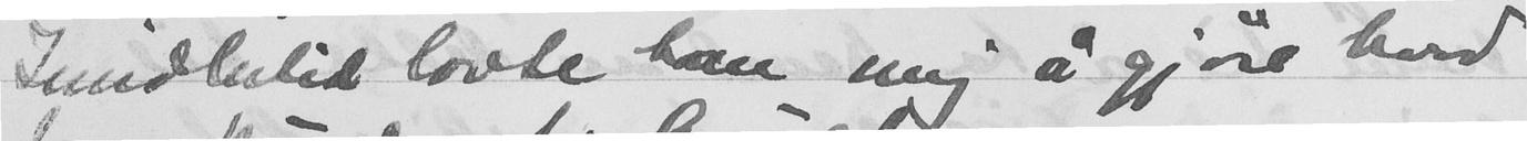Sundleitid lovte  mig å gjøre hvad
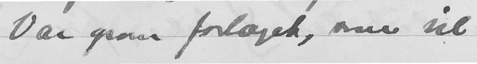Var opom forlaget, som vil
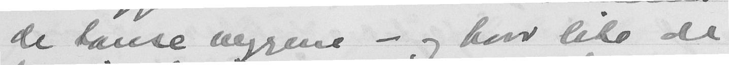de tause veggene - og hvor lite de
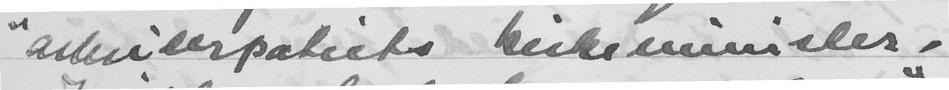"arbeiderpartiets kirkeminister,
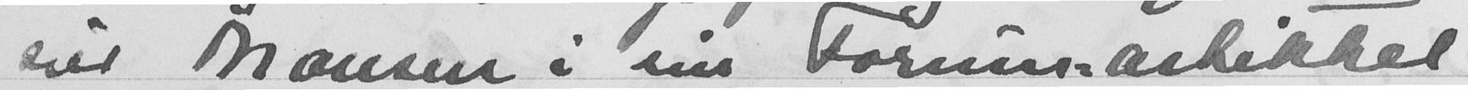sier Nansen i sin Forum-artikkel
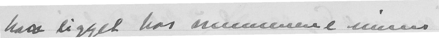har ligget hos mummiene innen
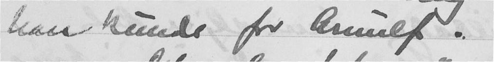han kunde for Arnulf.
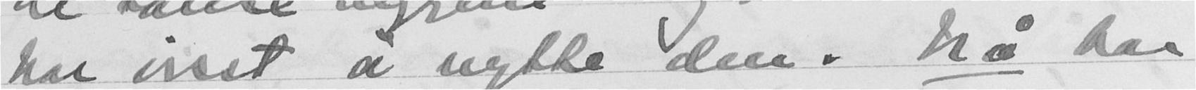har visst å nytte den. Nå har
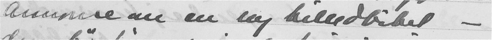Annonse om en ny billedbruket -
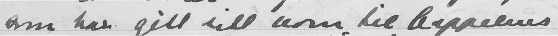som har gitt sitt navn til Cappelens
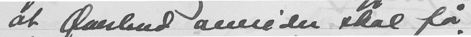at Øverland omsider skal få
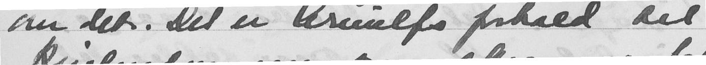om det. Det er Arnulfs forhold til
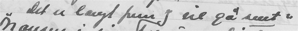"Det er langt frem og vil gå sent"
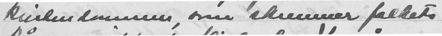kristendommen som skremmer folkets
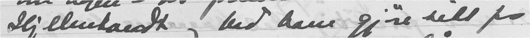Hjelmtveidt og bad ham gjøre sitt for
